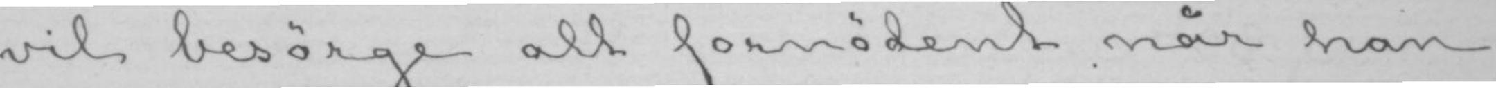vil besørge alt fornødent når han
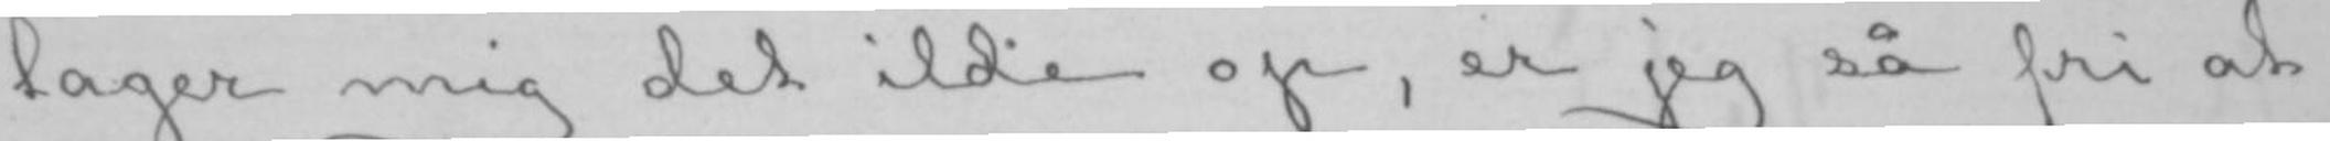tager mig det ilde op, er jeg så fri at
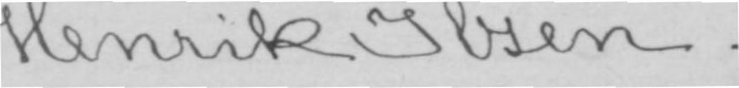Henrik Ibsen.
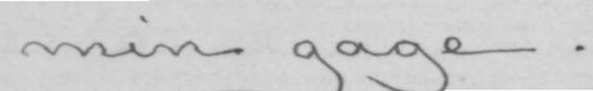i min gage.
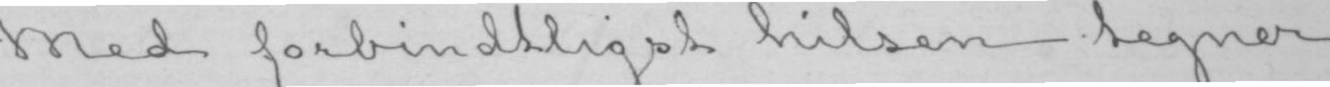Med forbindtligst hilsen tegner
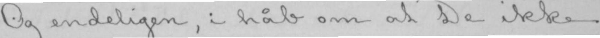Og endeligen, i håb om at De ikke
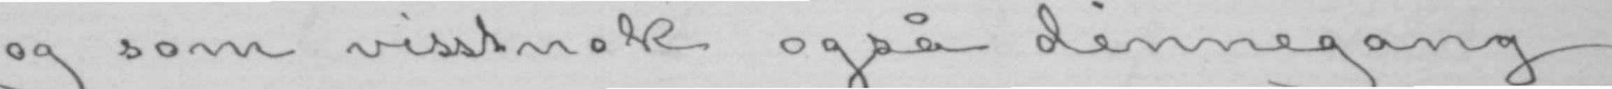og som visstnok også dennegang
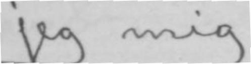jeg mig
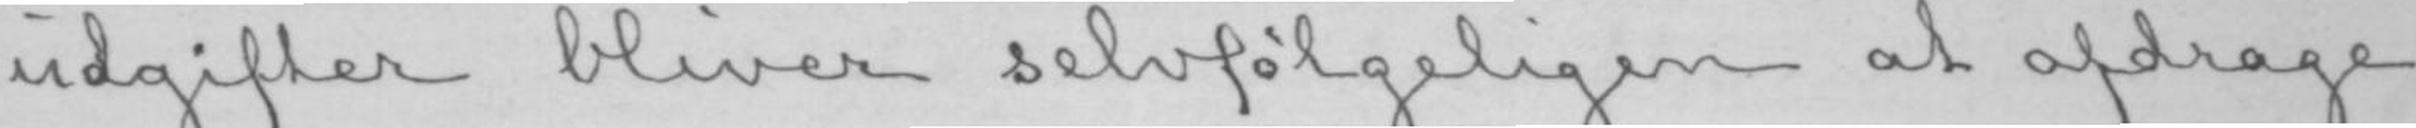udgifter bliver selvfølgeligen at afdrage
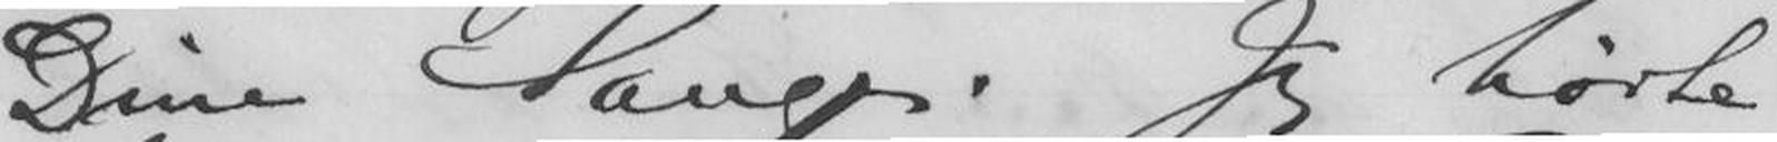Dine Sange. Jeg hørte
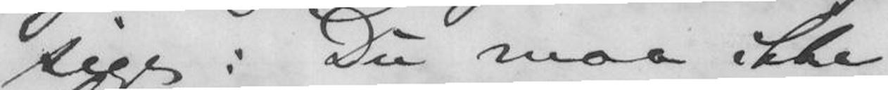sige: Du maa ikke
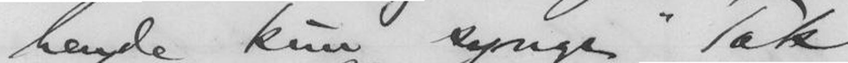hende kun synge "Tak
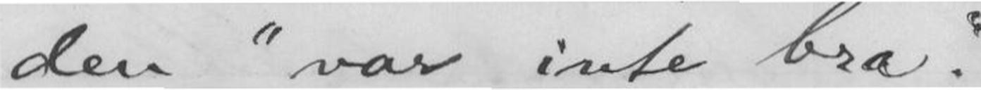den "var inte bra".
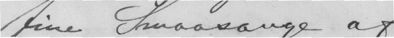fine Smaasange af
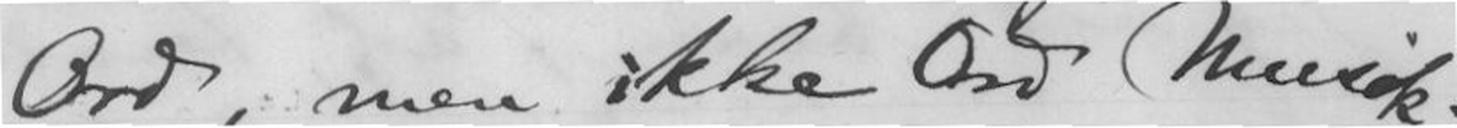Ord, men ikke Ord Musik.
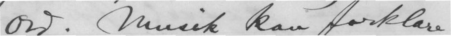Ord. Musik kan forklare
